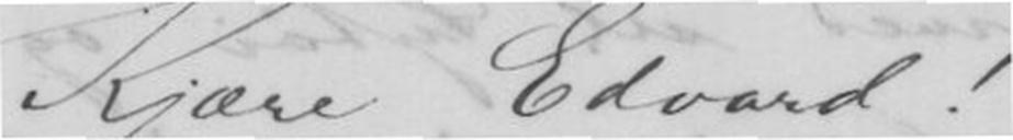Kjære Edvard!
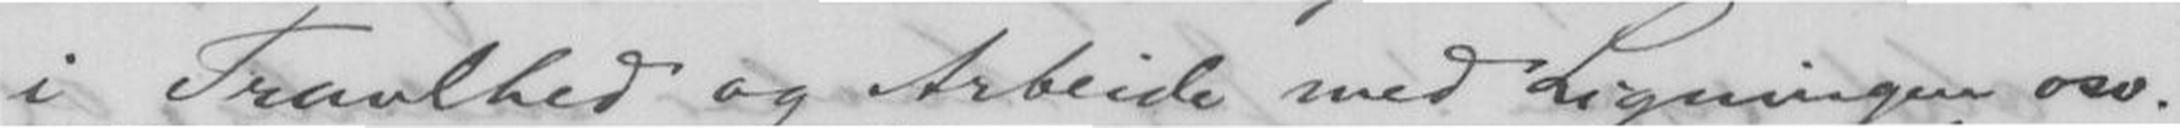i Travlhed og Arbeide med Ligningen
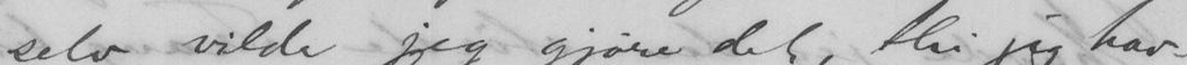selv vilde jeg gjøre det, thi jeg hav-
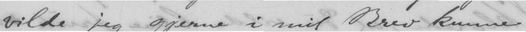vilde jeg gjerne i mit Brev kunne
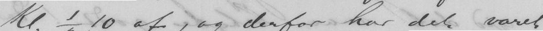Kl. ½ 10 af, og derfor har det varet
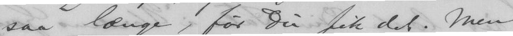saa længe, før Du fik det. Men
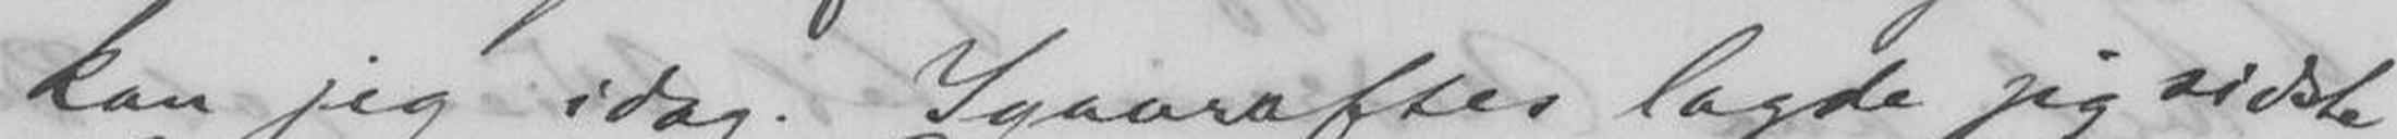kan jeg idag. Igaaraftes lagde jeg sidste
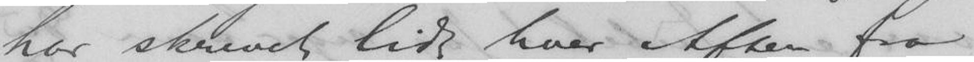har skrevet lidt hver Aften fra
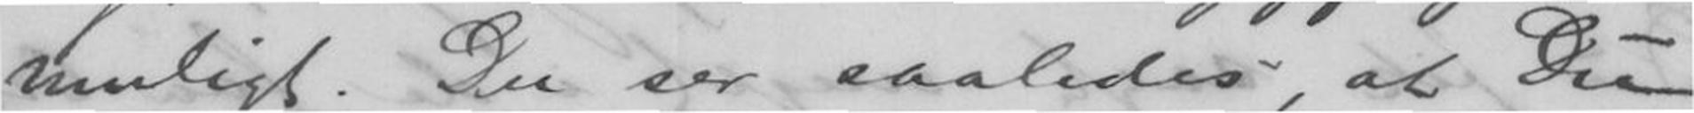muligt. Du ser saaledes, at Du
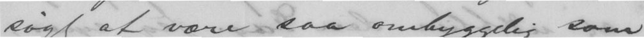søgt at være saa omhyggelig som
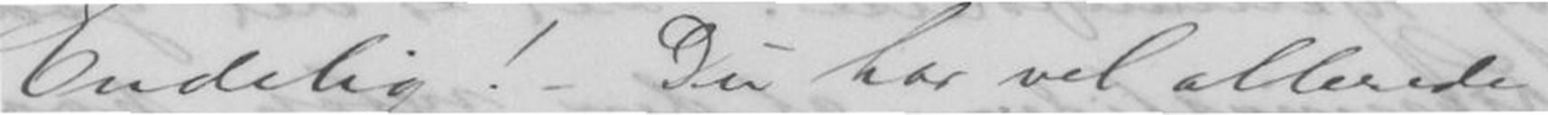Endelig! Du har vel allerede
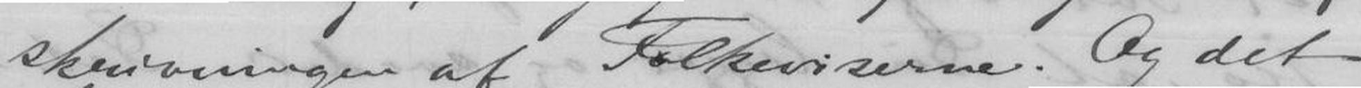skrivningen af Folkeviserne. Og det
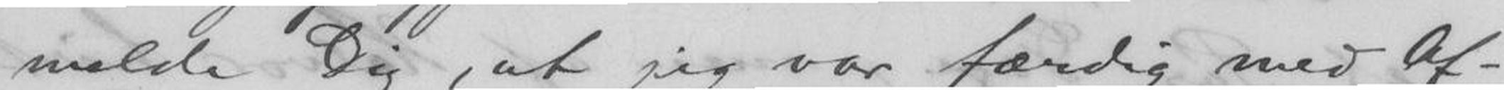melde Dig, at jeg var færdig med Af-
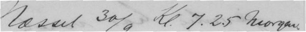Næsset 30/9 Kl. 7.25 Morgen
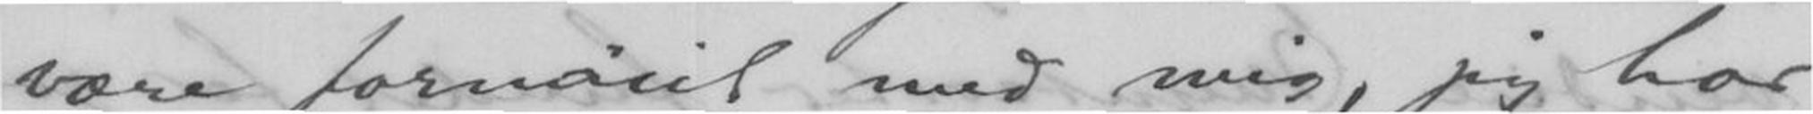være fornøiet med mig, jeg har
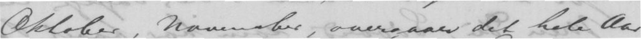Oktober, November, overgaar det hele Aar
